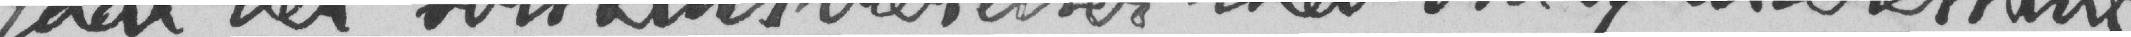faar der solskinsværelser med vid og interessant
